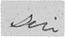sin
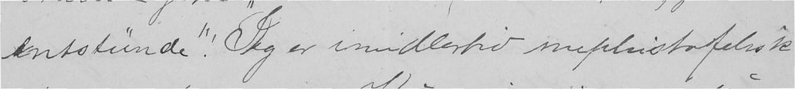entstunde"! Jeg er imidlertid mephistofelisk
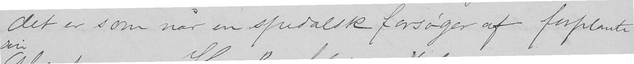det er som når en spedalsk forsøger af forplante
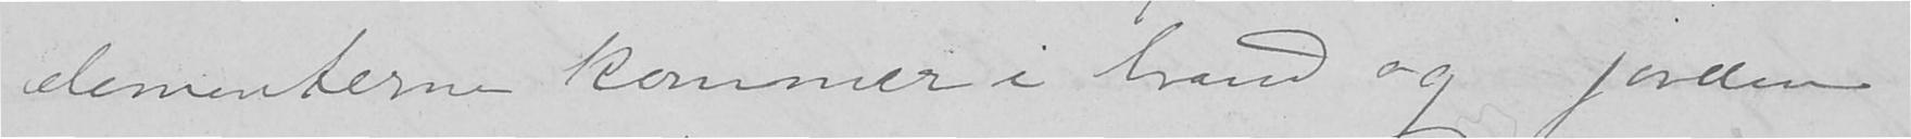elementerne kommer i brand og jorden
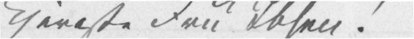Kjereste Fru Ibsen!
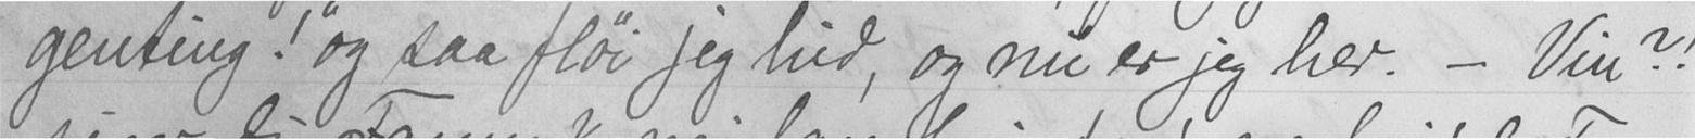genting!" og saa fløi jeg hid, og nu er jeg her. - Vin?!
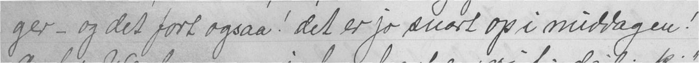ger - og det fort ogsaa! det er jo snart op i middagen!
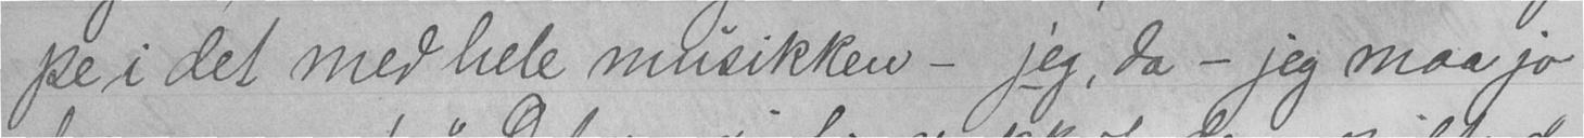pe i det med hele musikken - jeg, da - jeg maa jo
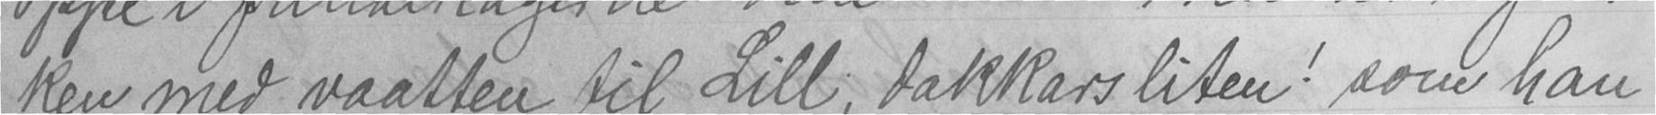ken med vaatten til Lill, dakkars liten! som han
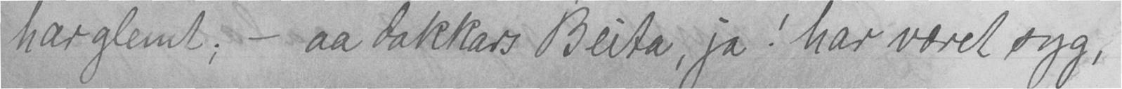har glemt; - aa dakkars Beita, ja! har været syg,
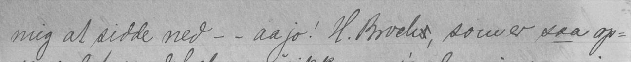mig at sidde ned - - aa jo! H. Brochs, som er saa op-
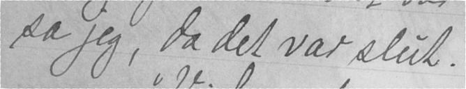sa jeg, da det var slut.
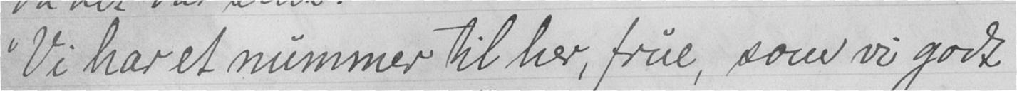"Vi har et nummer til her, frue, som vi godt,
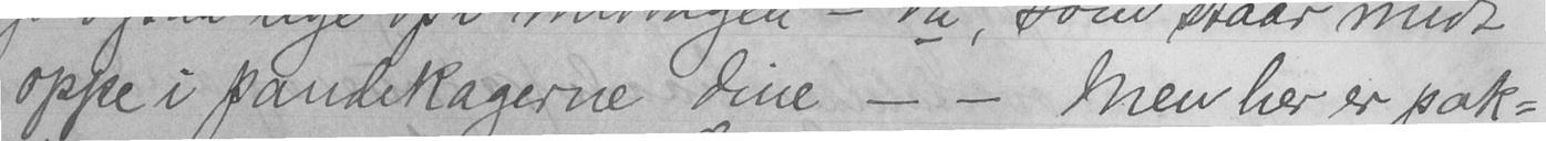oppe i pandekagerne dine - - Men her er pak-
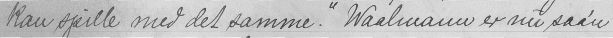kan spille med det samme." Waalmann er nu saa'n
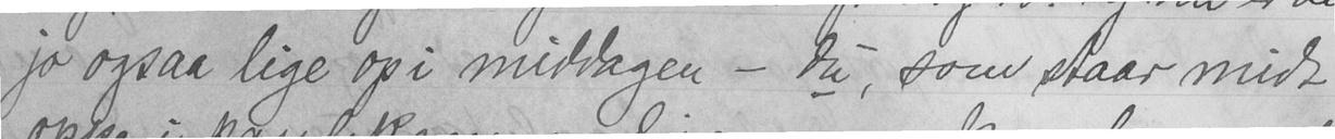jo ogsaa lige op i middagen - du, som staar midt
